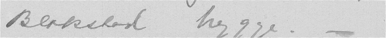Blakstad brygge.
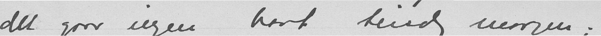det gaar ingen baat tirsdag morgen;
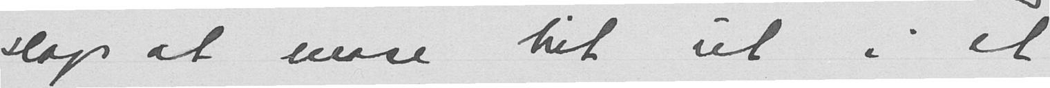slap at mase hit ut i et
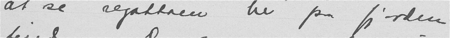at se regattaen her paa fjorden
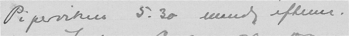Piperviken 5.30 mandag efterm.
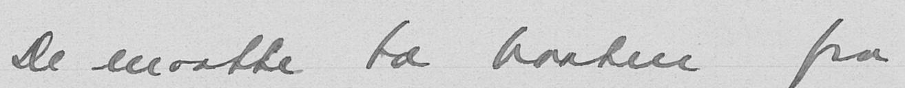De maatte ta baaten fra
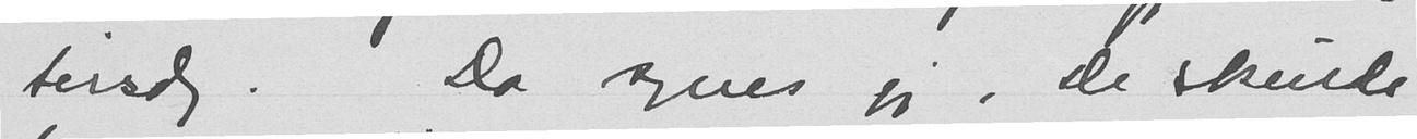tirsdag. Da synes jeg, De skulde
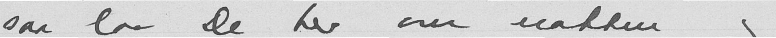saa laa De her om natten og
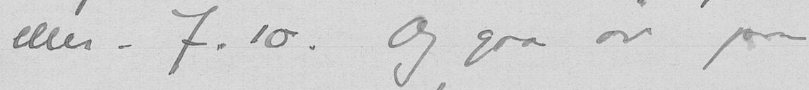eller - 7.10. Og gaa av paa
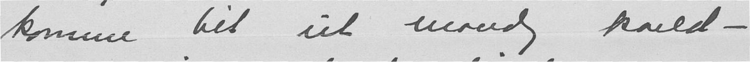komme hit ut mandag kveld -
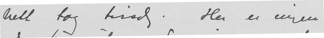hett tog tirsdag. Her er ingen
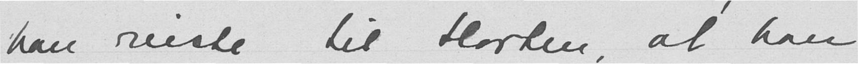han reiste til Horten, at han
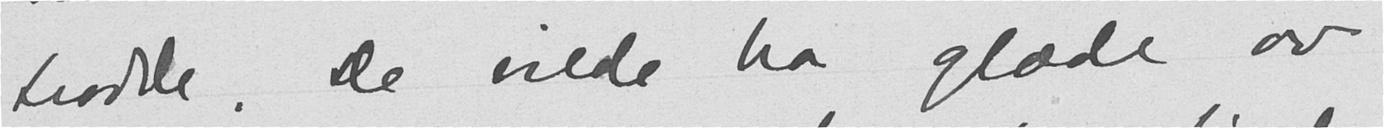trodde, De vilde ha glæde av
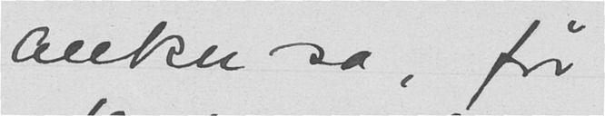Anker sa, før
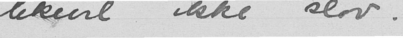likevel ikke sløv.
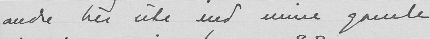andre her ute end mine gamle
